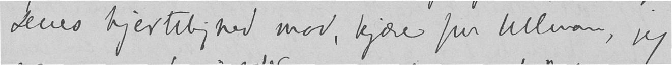Deres hjertelighed mod, kjære fru Ullman, jeg
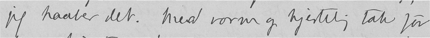jeg haaber det. Med varm og hjertelig tak for
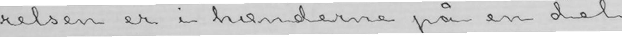relsen er i hænderne på en del
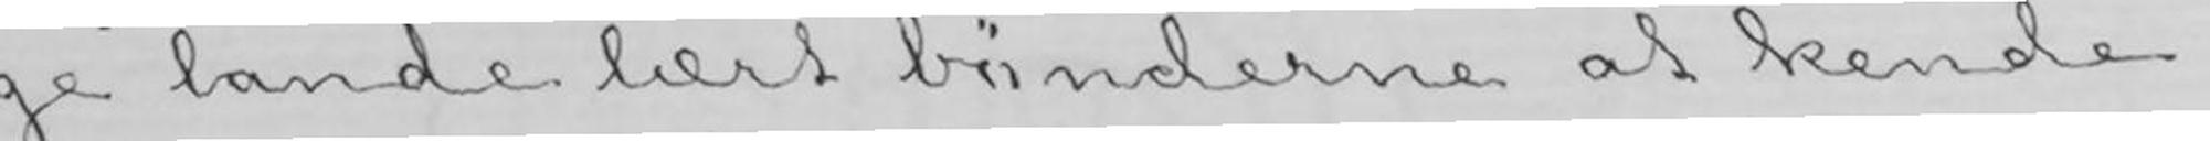ge lande lært bønderne at kende;
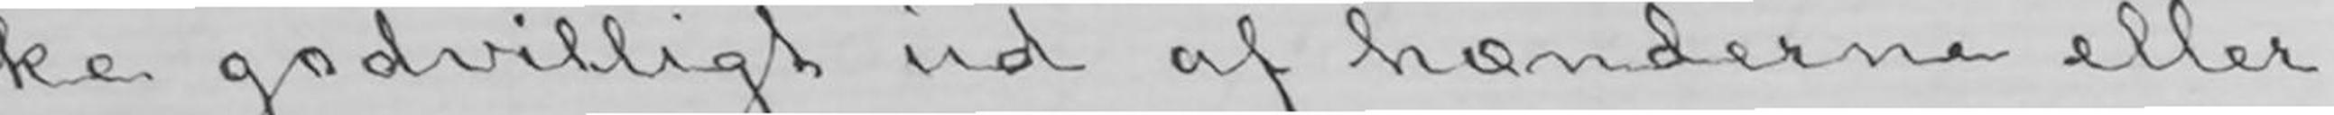ke godvilligt ud af hænderne eller
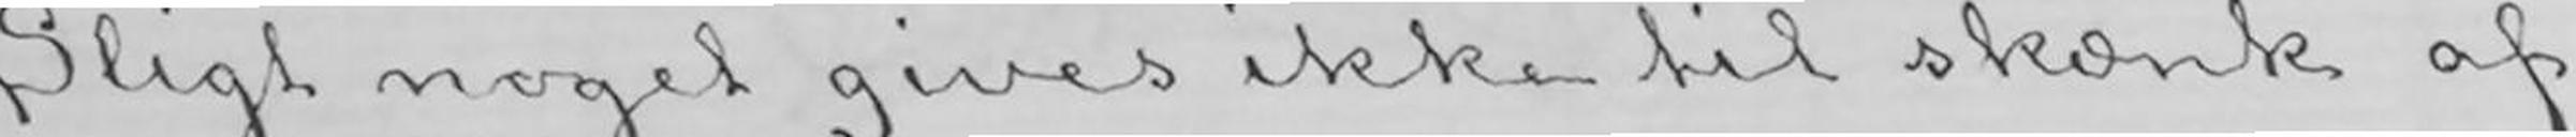Sligt noget gives ikke til skænk af
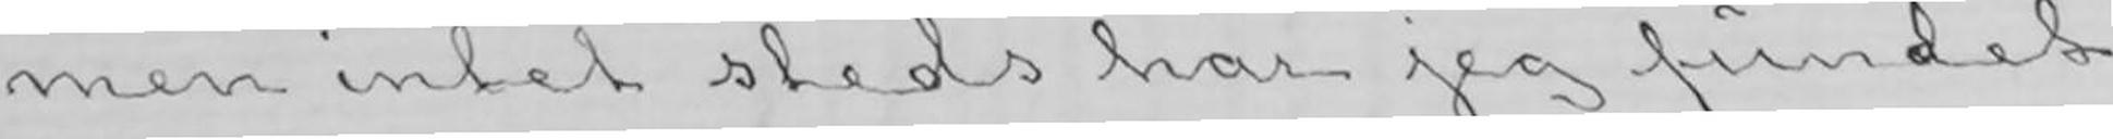men intet steds har jeg fundet
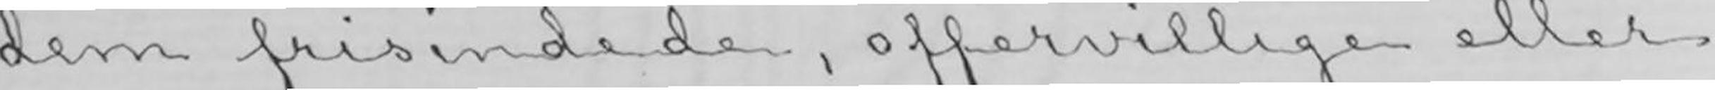dem frisindede, offervillige eller
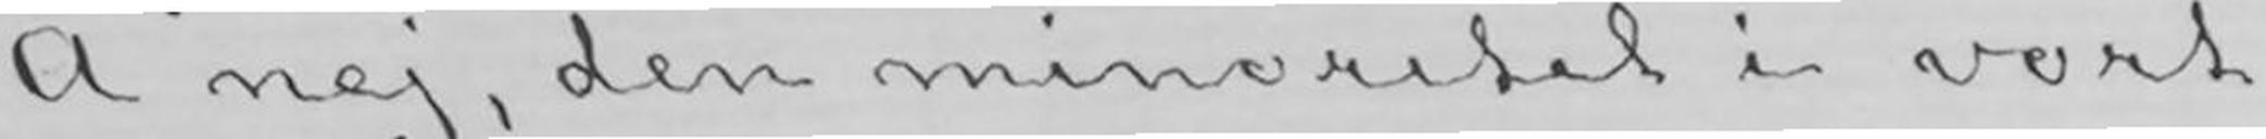Å nej, den minoritet i vort
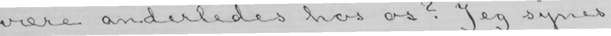være anderledes hos os? Jeg synes
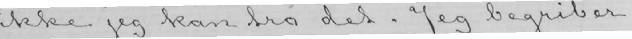ikke jeg kan tro det. Jeg begriber
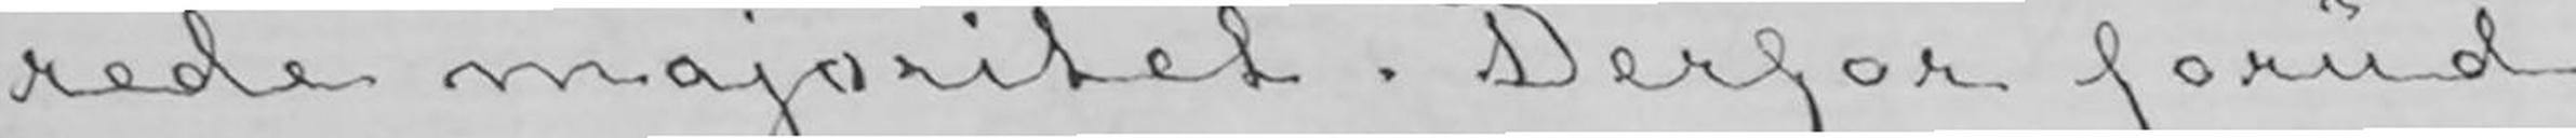rede majoritet. Derfor forud-
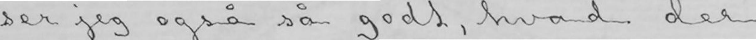ser jeg også så godt, hvad der
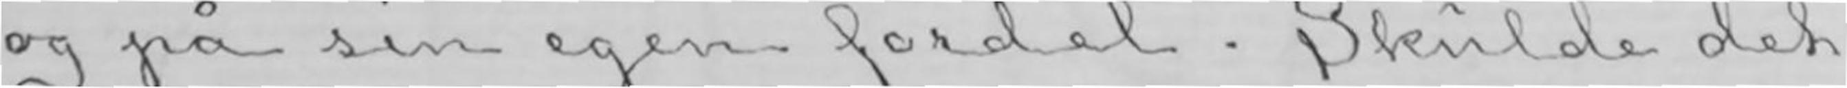og på sin egen fordel. Skulde det
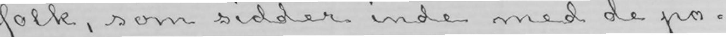folk, som sidder inde med de po-
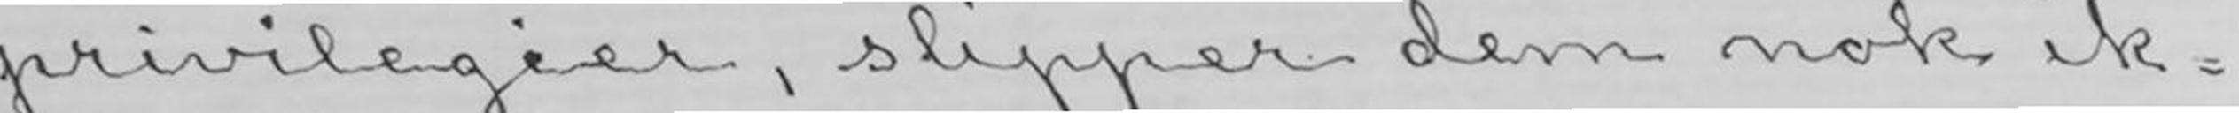privilegier, slipper dem nok ik-
